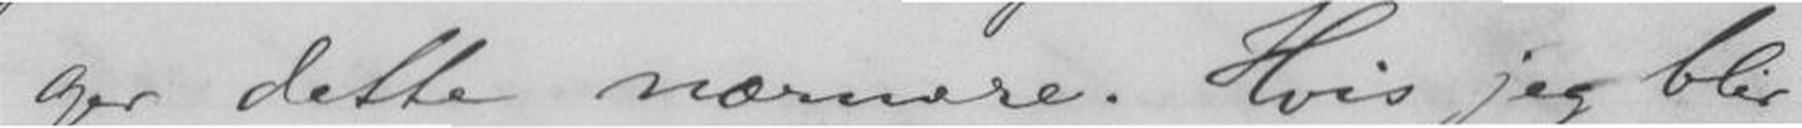ger dette nærmere. Hvis jeg blir
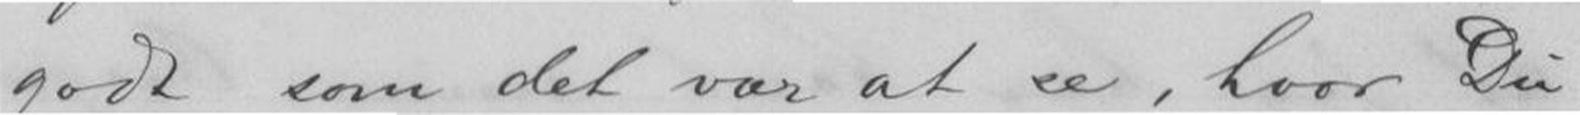godt som det var at se, hvor Du
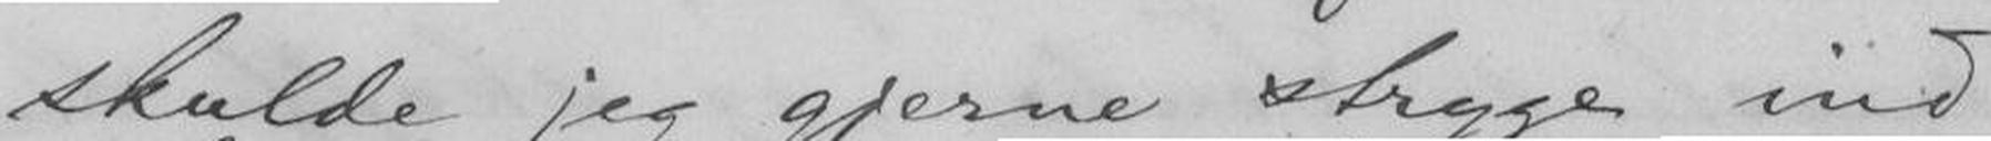skulde jeg gjerne stryge ind
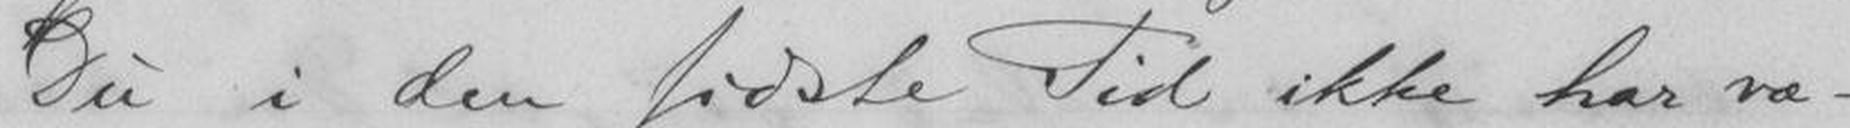Du i den sidste Tid ikke har væ-
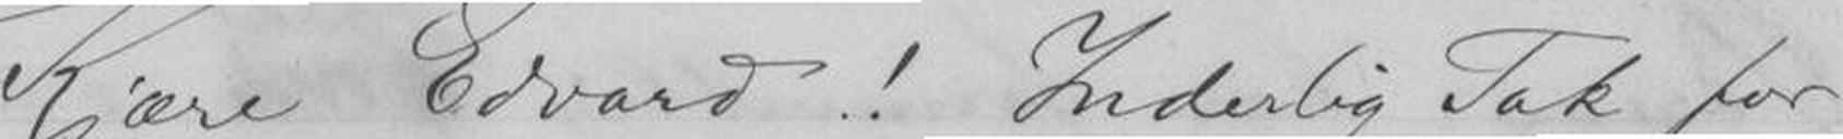Kjære Edvard! Inderlig Tak for
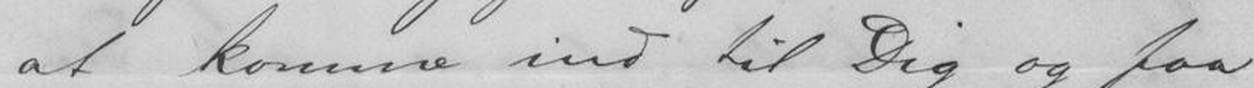at komme ind til Dig og faa
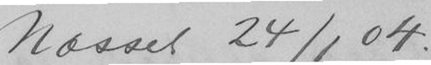Næsset 24/1 04.
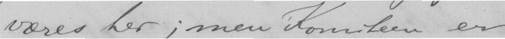væres her; men Komiteen er
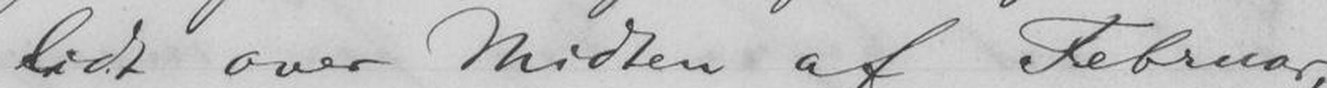lidt over Midten af Februar,
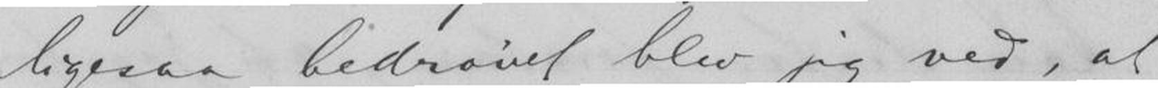ligesaa bedrøvet blev jeg ved, at
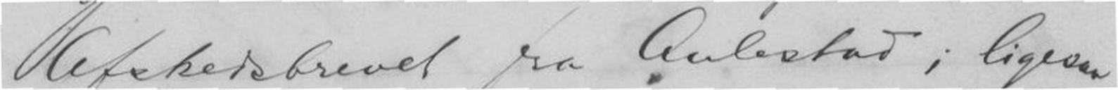Afskedsbrevet fra Aulestad; ligesaa
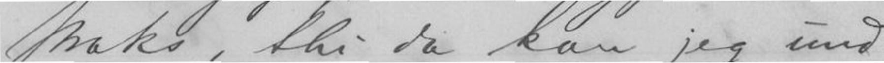straks, thi da kan jeg und-
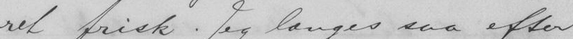ret frisk. Jeg længes saa efter
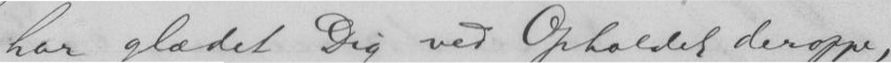har glædet Dig ved Opholdet deroppe,
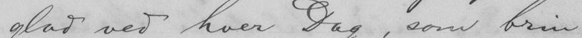glad ved hver Dag, som brin-
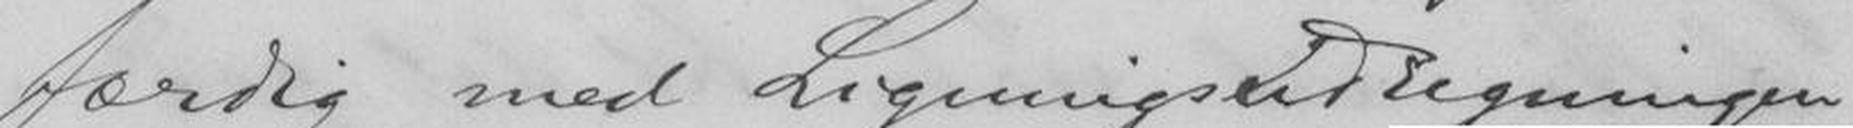færdig med Ligningsudregningen
